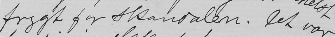frygt for skandalen. Det var
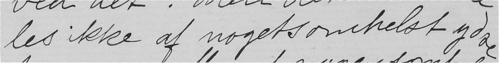les ikke af nogetsomhelst ydre
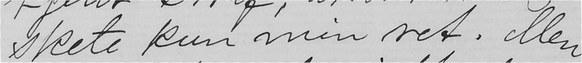skete kun min ret. Men
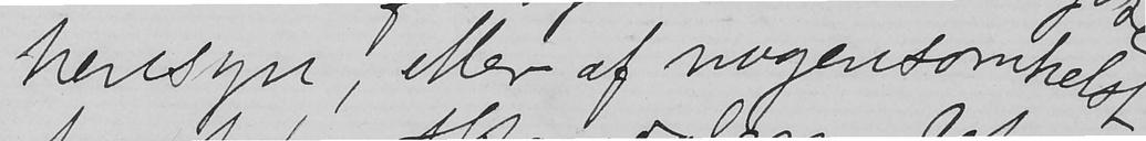hensyn, eller af nogensomhelst
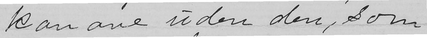kan ane uden den, som
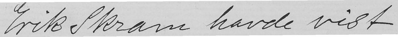Erik Skram havde vist
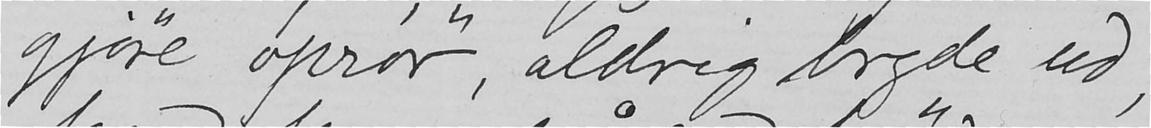gjøre oprør, aldrig bryde ud,
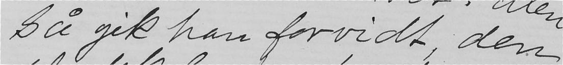så gik han forvidt, den
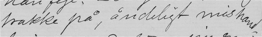trakke på, åndeligt mishand-
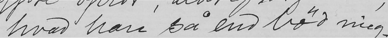hvad han så end bød mig.
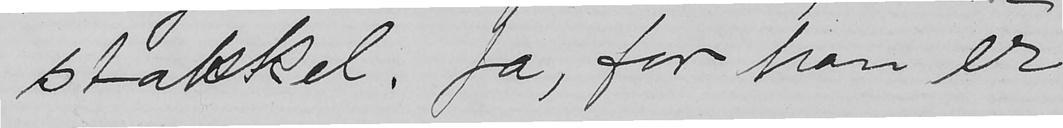stakkel. Ja, for han er
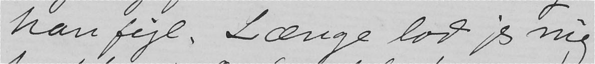han fejl. Længe lod jeg mig
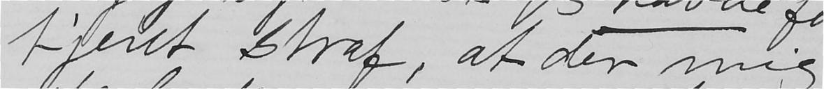tjent straf, at der mig
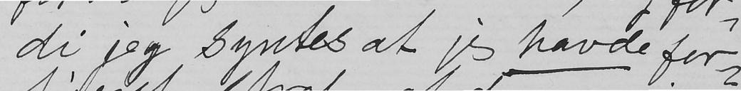di jeg syntes at jeg havde for-
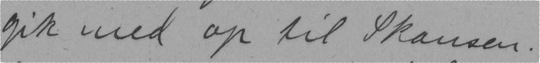gik med op til Skansen.
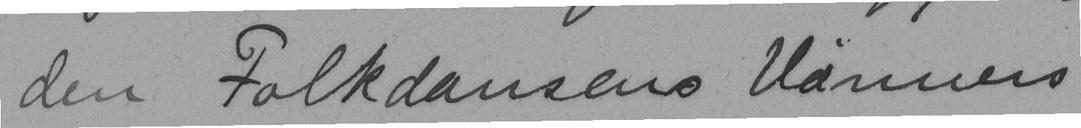den Folkdansens Vänners
